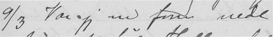9/3 Var jeg om form nede
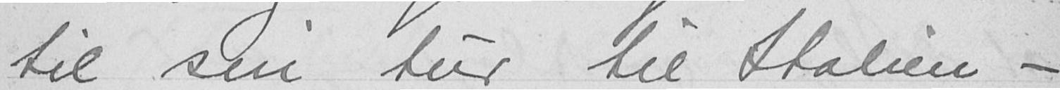til sin tur til Stalien -
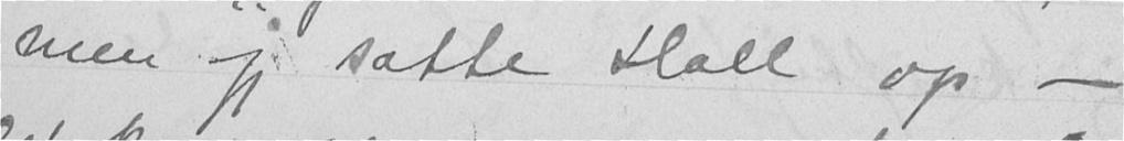men jeg satte Hall op -
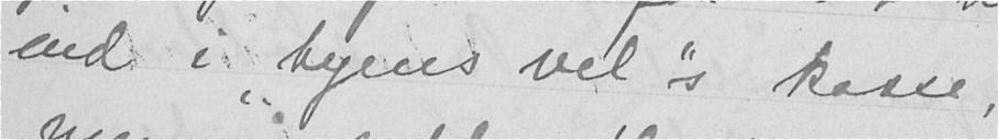ind i "byens vel"s  kasse,
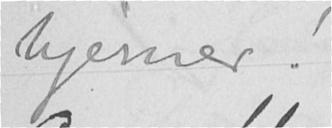hjerner!
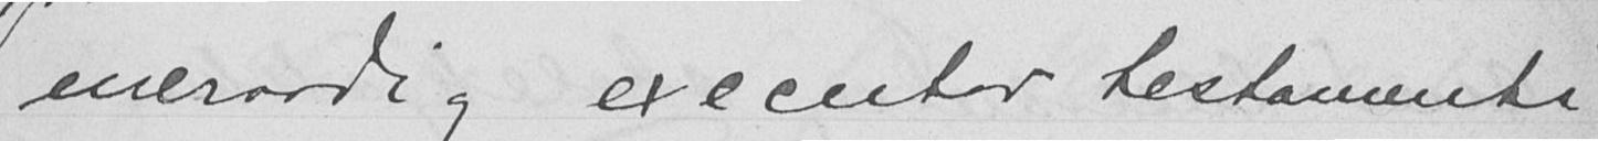eneraadig executor testamenti
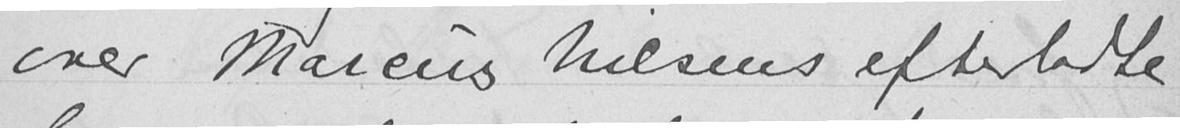over Marcus Nilsens efterladte
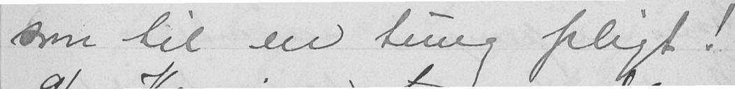som til en tung pligt!
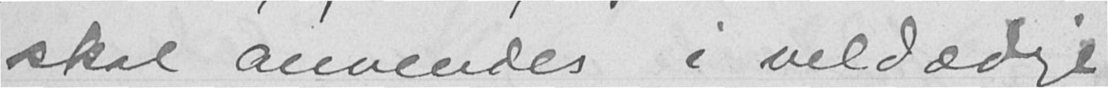skal anvendes i veldædige
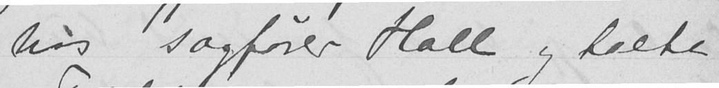hos sagfører Hall og talte
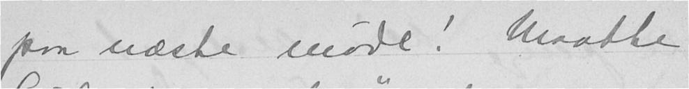paa næste møde! Maatte
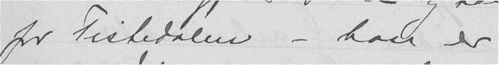for Tistedalen - han er
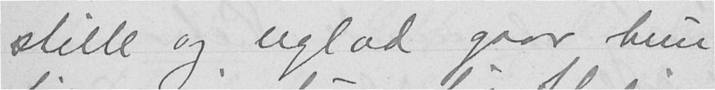stille og uglad gaar hun
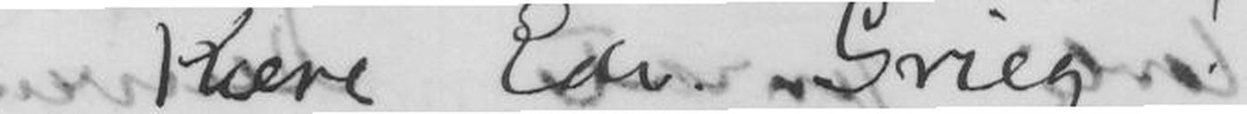Kære Edv. Grieg!
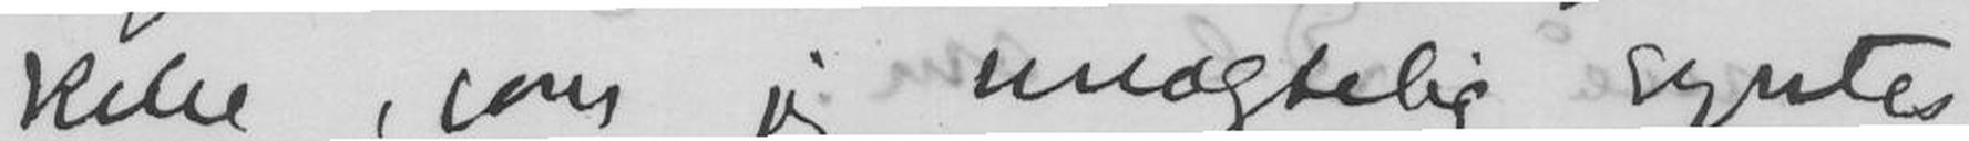kelse, som jeg unægtelig syntes
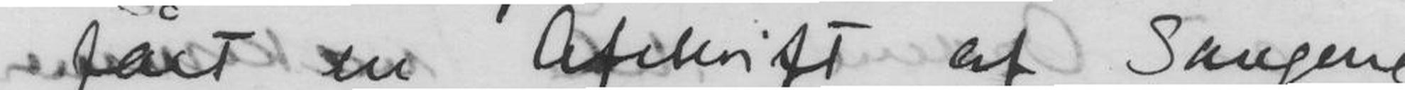fået en Afskrift af Sangene
